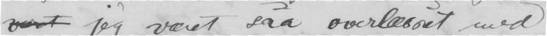været jeg været saa overlæsset med
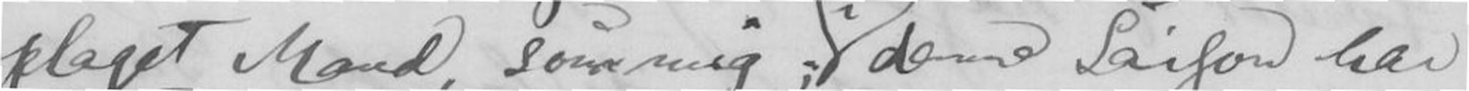plaget Mand, som mig; i denne Saison har
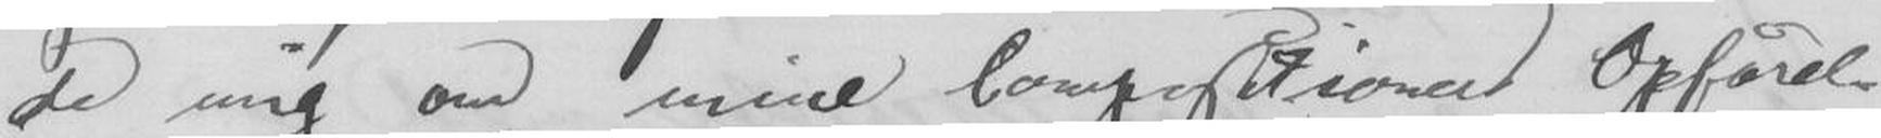de mig om mine Compositioner Opførel-
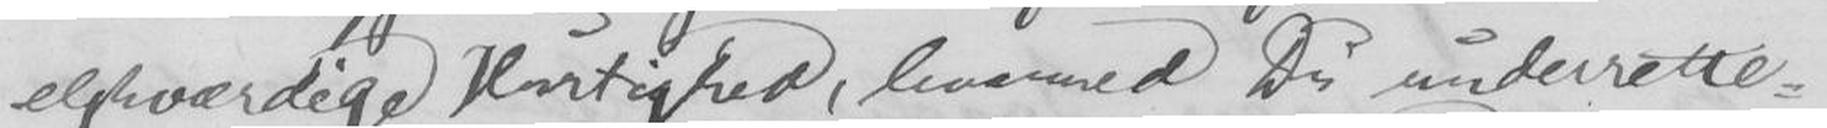elskværdige Hurtighed, hvormed Du underrette-
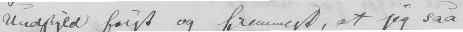Undskyld først og fremst, at jeg saa
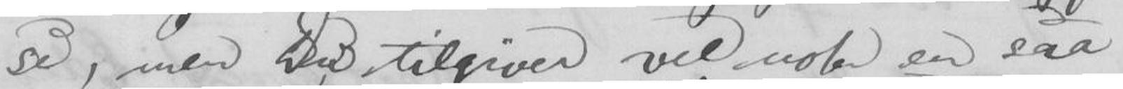se, men Du tilgiver vel nok en saa
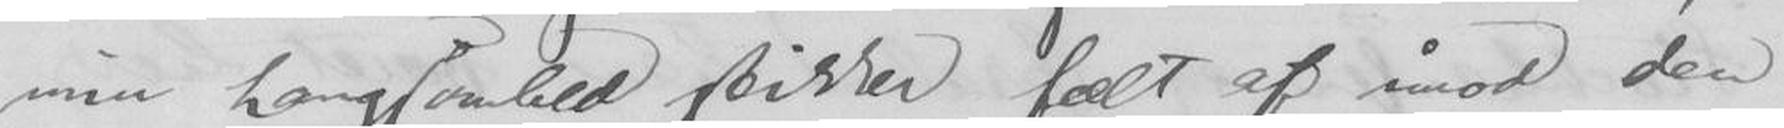min Langsomhed stikker fælt af imod den
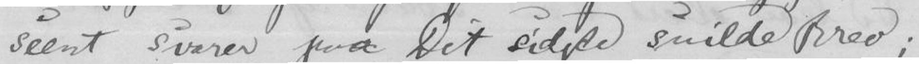seent svarer paa Dit sidste snilde Brev,
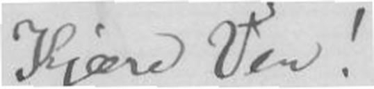Kjære Ven!
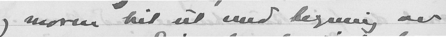og moren hit ut med tegning av
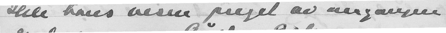Hele hans vesen preget av omgangen
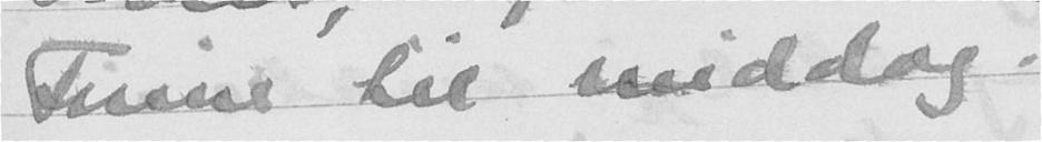Finne til middag.
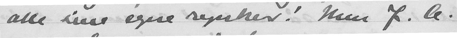alle sine egne rynker. Men J. C.
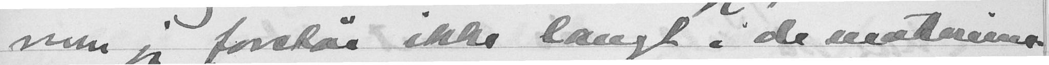men jeg forstår ikke langt i de materiene.
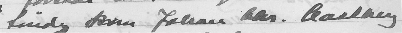Søndag kom Johan Chr. Castberg
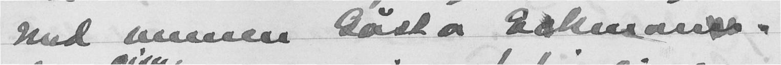med vennen Gøsta Eckmann.
